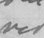ved
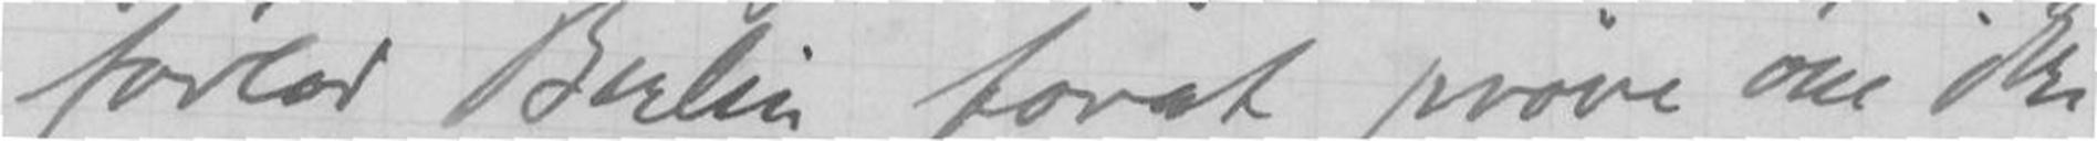forlot Berlin forat prøve om ikke
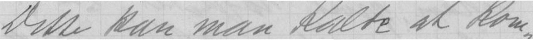Dette kan man Kalde at Kom-
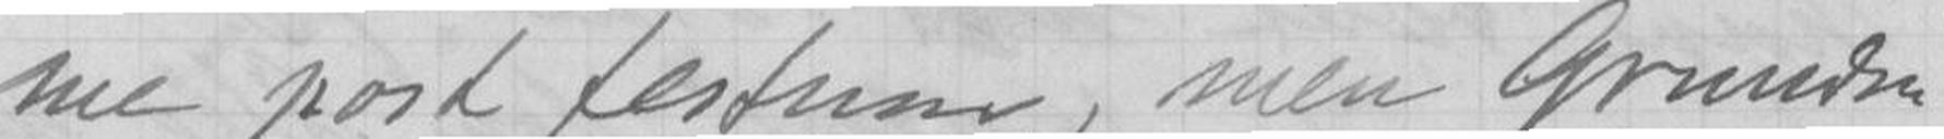me post festum, men Grunden
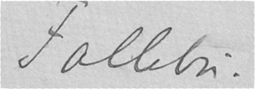Follebu.
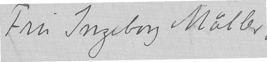Fru Ingeborg Møller,
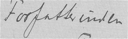Forfatterinden
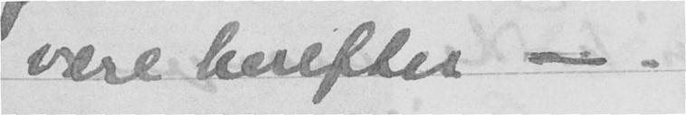være herefter - .
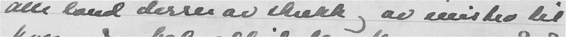alle land dirrer av skrekk og av mistro til
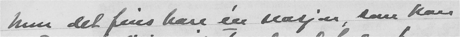Men det fins bare en nasjon som kan
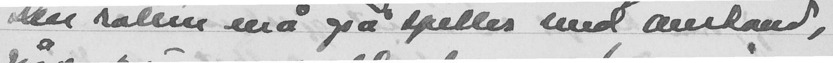Den rollen må også spilles med Aneland,
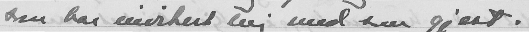som har invitert sig med som gjort.
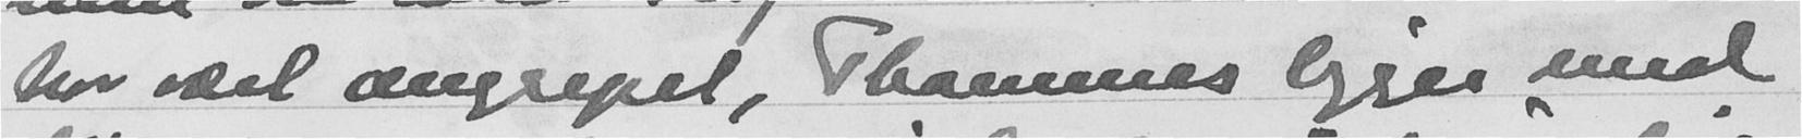har vært angrepet, Thommes ligger med
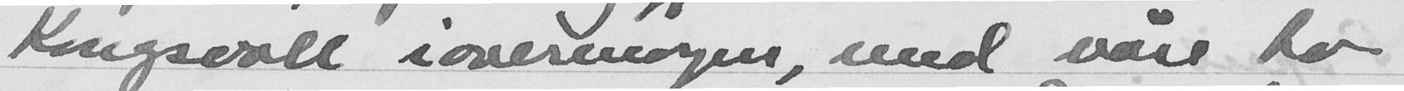Kongsvoll iovermorgen, med våre to
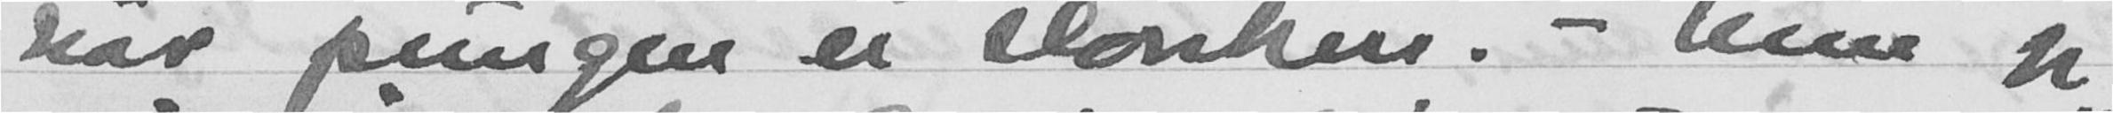når pungen er slanken. - Men jeg
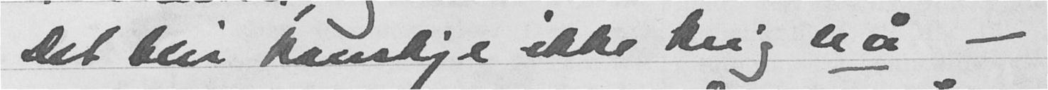Det blir kanskje ikke krig nå -
